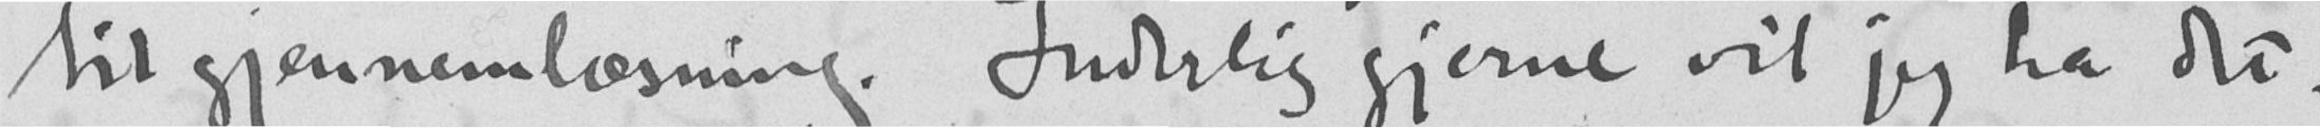til gjennemlæsning. Inderlig gjerne vil jeg ha det.
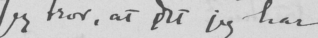Jeg tror, at det jeg har
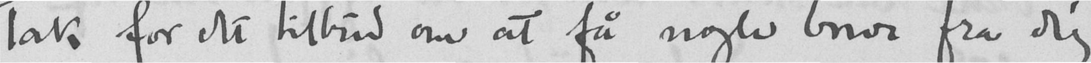Tak for det tilbud om at få nogle breve fra dig
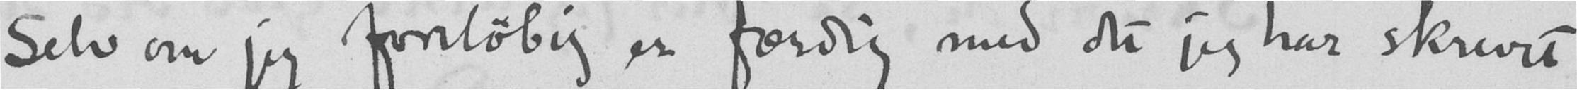Selv om jeg foreløbig er ferdig med det jeg har skrevet
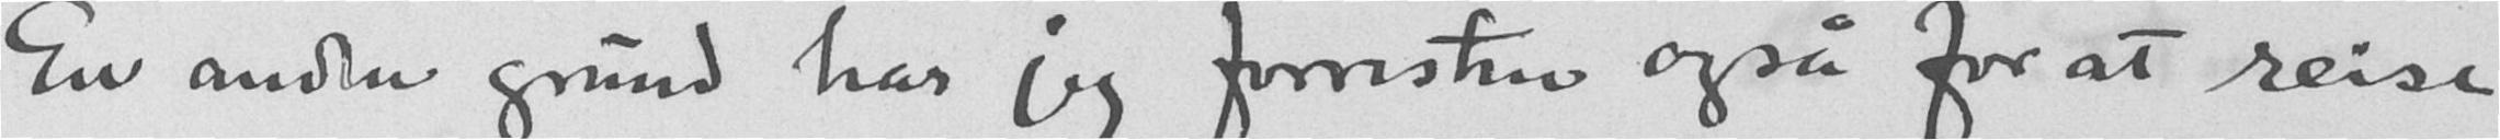En anden grund har jeg forresten også for at reise
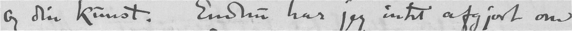og din kunst. Endnu har jeg intet afgjort om
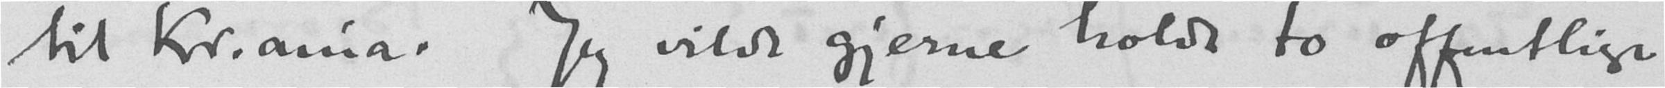til Kr.ania. Jeg vilde gjerne holde to offentlige
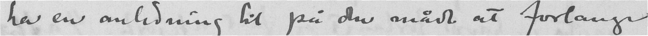ha en anledning til på den måde at forlange
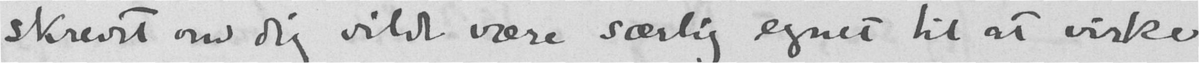skrevet om dig vilde være særlig egnet til at virke
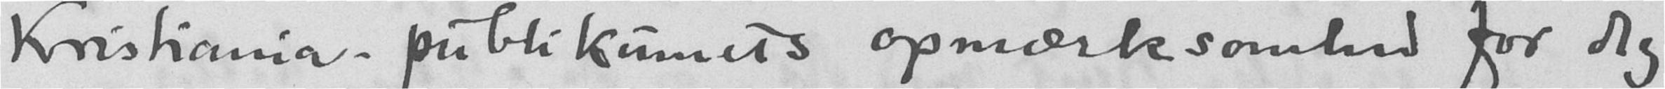Kristiania- publikumets opmærksomhed for deg
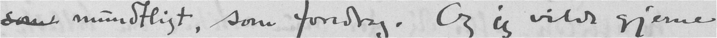som mundtligt, som foredrag. Og jeg vilde gjerne
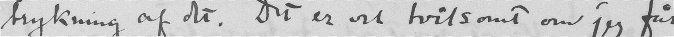trykning af det. Det er vel tvilsomt om jeg får
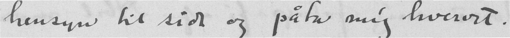hensyn til side og påta mig hvervet.
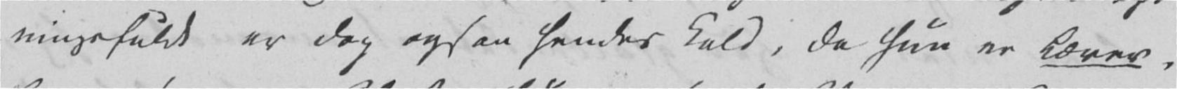ningsfuldt er dog ogsaa hendes Kald, da hun er Lærer,
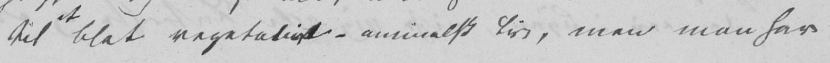til et blot vegetativt-animalsk Liv, men man har
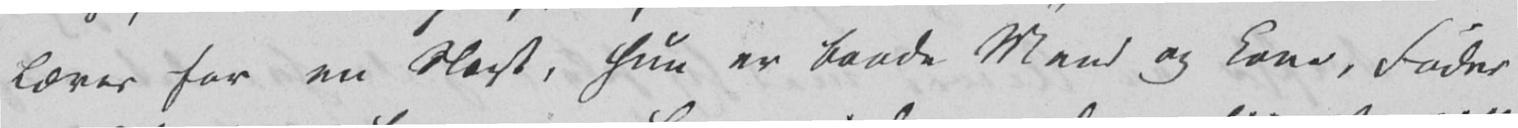Lærer for en Slægt, hun er baade Mand og Kone, Fader
